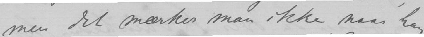men det mærkes ikke naar han
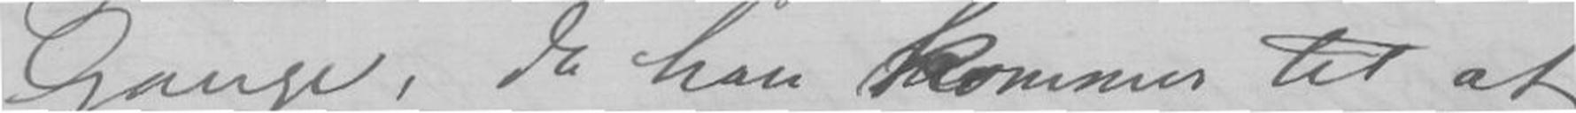Gange, da han kommer til at
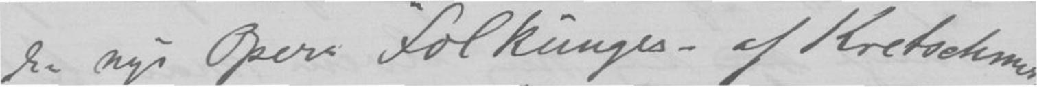da nye Opera "Folkunges- af Kretschmer,
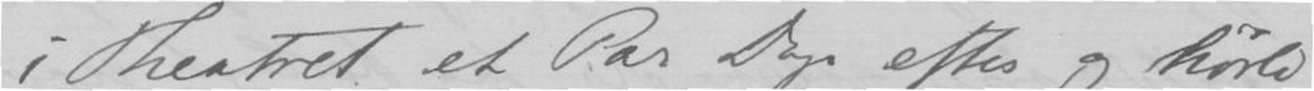i Theatret et Par Dage efter og hørte
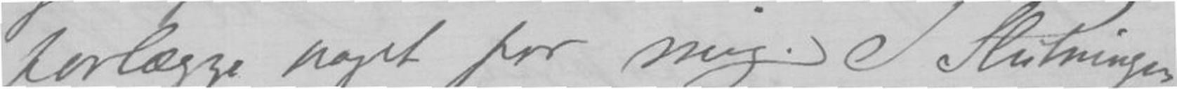forlægge noget for mig. I Slutningen
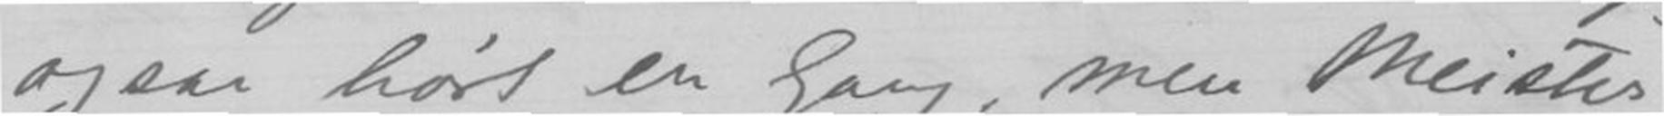ogsaa hørt en Gang, men Meister
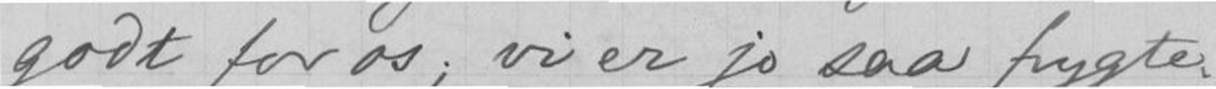godt for os; vi er jo saa frygte-
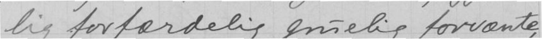lig forfærdelig gruelig forvænte,
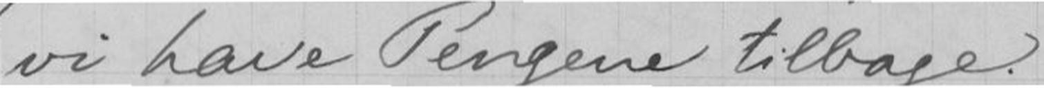vi have Pengene tilbage.
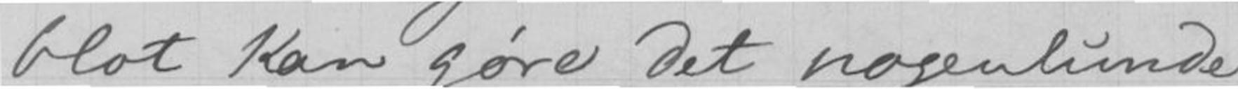blot kan gøre det nogenlunde
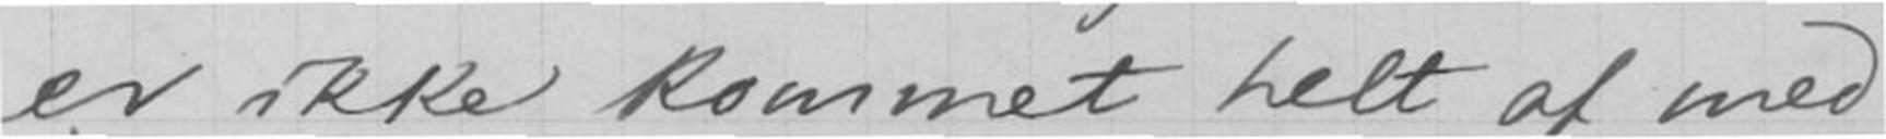er ikke kommet helt af med
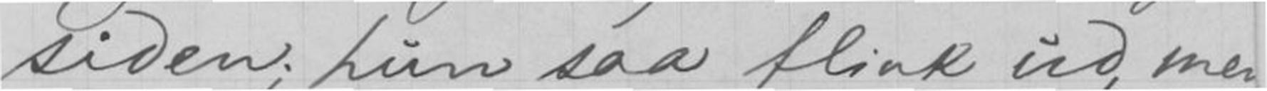siden; hun saa flink ud, men
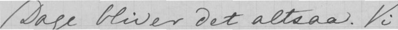Dage bliver det altsaa. Vi
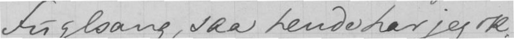Fuglsang, saa hende har jeg ik-
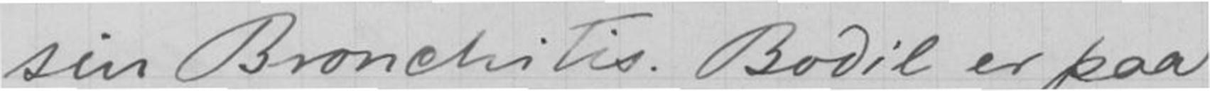sin Bronchitis. Bodil er paa
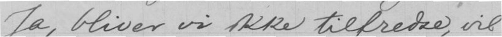Ja, bliver vi ikke tilfredse, vil
